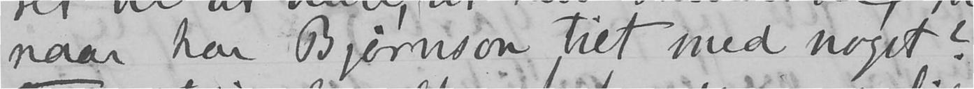naar har Bjørnson tiet med noget?
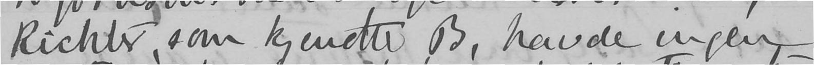Richter, som kjendte B, havde ingen
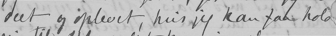seet og oplevet, hvis jeg kan faa hold-
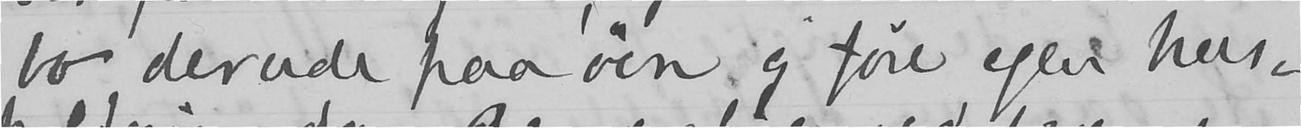bo derude paa øen og føre egen hus-
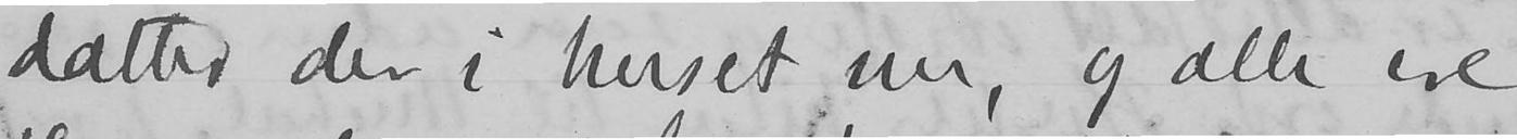datter der i huset nu, og alle ere
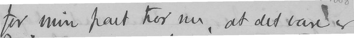for min part tror nu, at det bare er
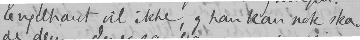Engelhardt vil ikke, og han kan nok ska-
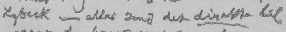Lybeck - eller send det direkte til
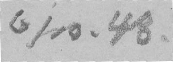6/10. 48.
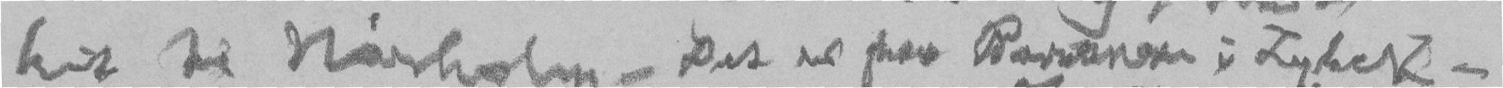hit til Nørholm. Det er fra Baronen i Lybeck -
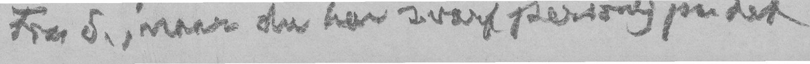Fru S., naar du har svart personlig paa det
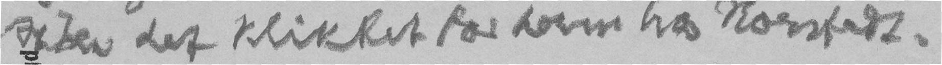siden det klikket for ham hos Norstedt.
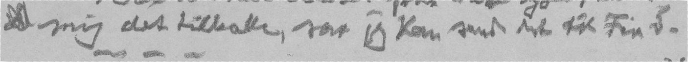d mig det tilbake, saa jeg kan sende det til Fru S.
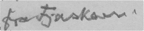fra Fjaskoen.
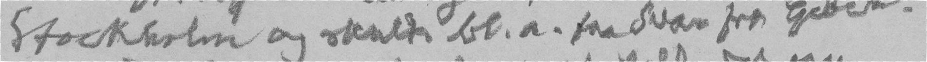Stockholm og skulde bl.a. faa Svar fra Geber.
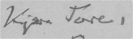Kjære Tore,
